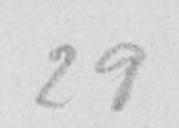29
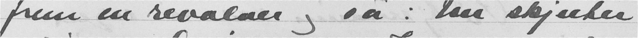frem en revolver og sa: Nu skjuter
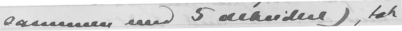sammen med 5 arbeidere), tok
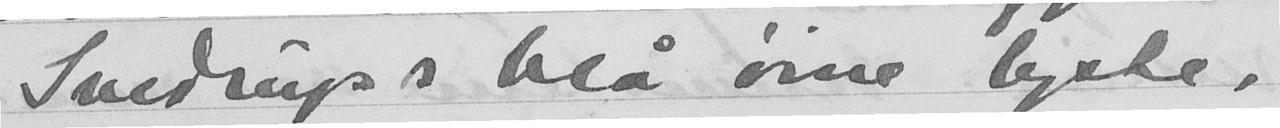Svedrup's blå øine lyste,
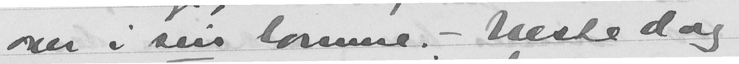over i sin lomme - Neste dag
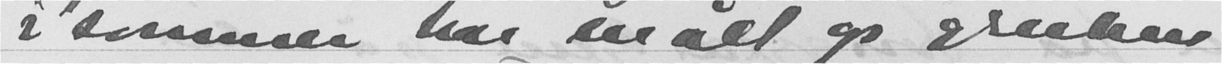i sommer har målt op gruber
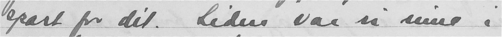spart for det. Siden var vi inne i
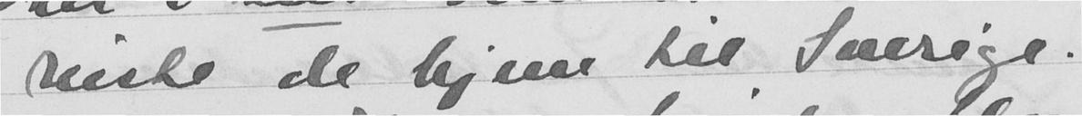reiste de hjem til Sverige.
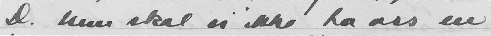D. Men skal vi ikke ha oss en
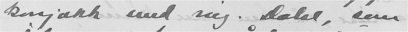konjakk med ing. Dahl, som
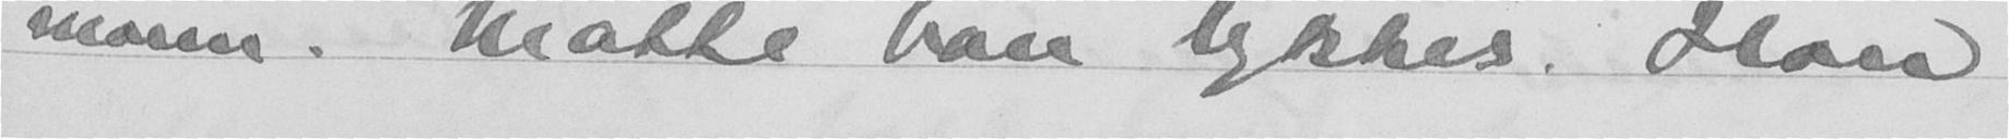mann. Måtte han lykkes. Han
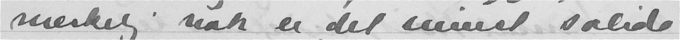merkelig nok er det minst solide
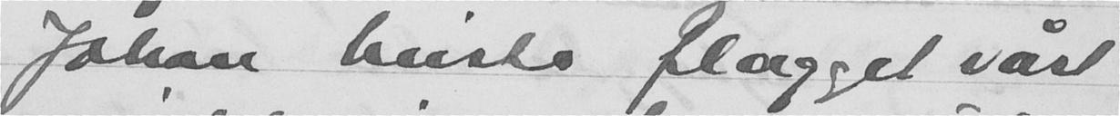Johan heiste flagget vårt
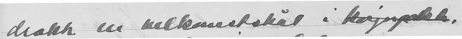drakk en velkomstskål i .
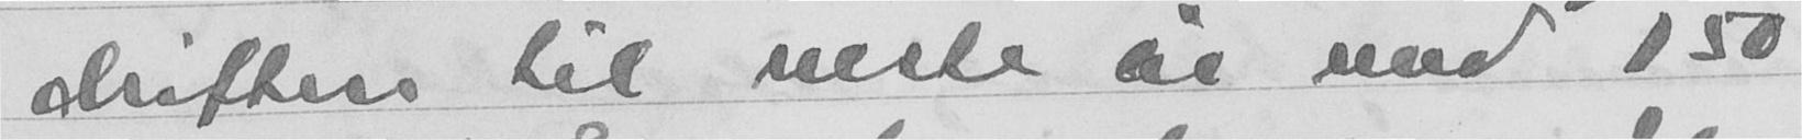driften til neste år med 150
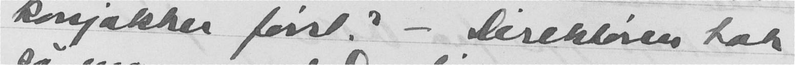konjakker først? - Direktøren tok
